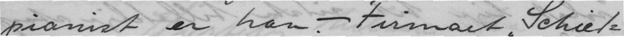pianist er han. - Firmaet "Schied-
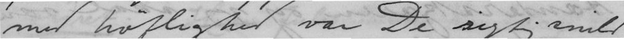med höflighed var De rigtig snild,
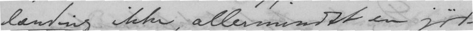lænding ikke, allermindst en jöde,
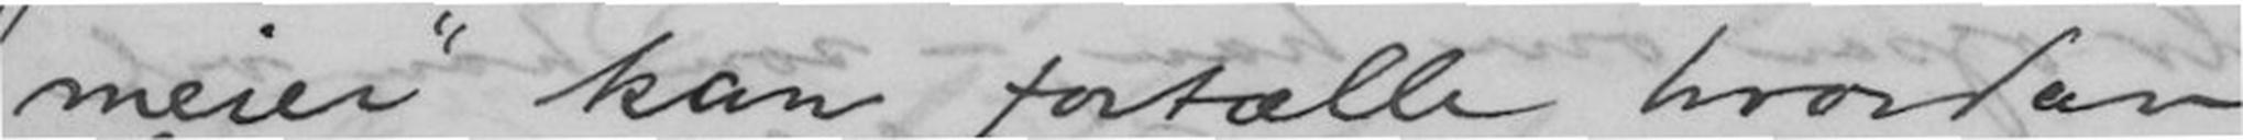meier" kan fortælle hvordan
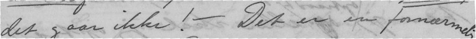det gaar ikke! -Det er en fornærmelig
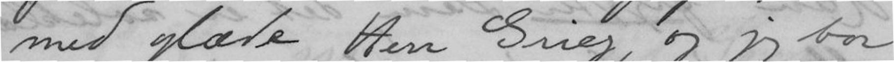med glæde Herr Grieg, og jeg bor
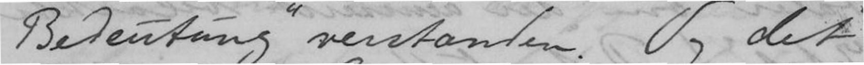Bedeutung" verstanden. Og det
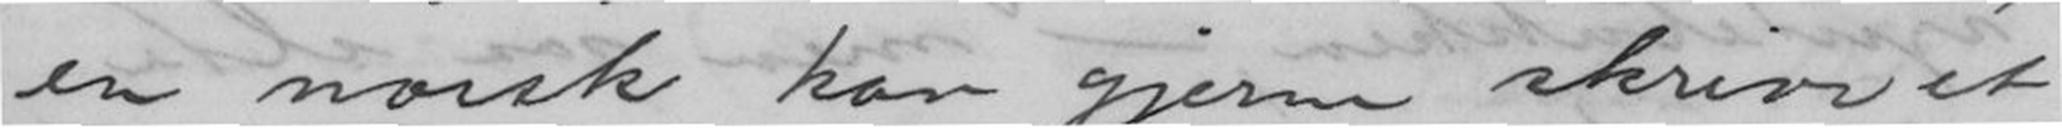en norsk kan gjerne skrive et
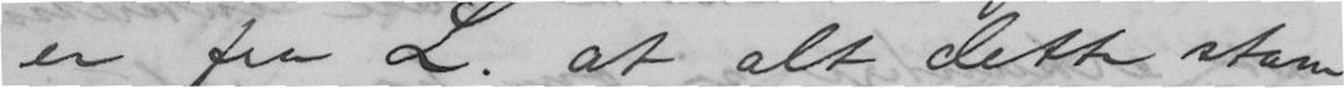er fra L. at alt dette stam
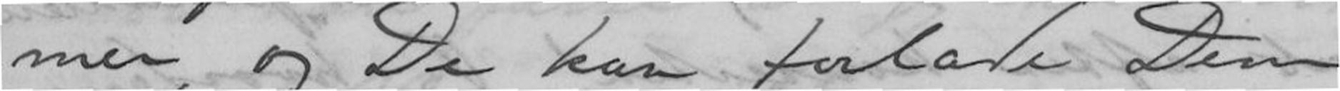mer, og De kan forlade Dem
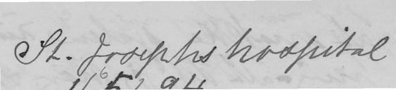St. Josephs hospital
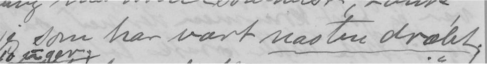jeg som har vært nasten dræbet.
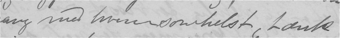gang med hvensomhelst, tænk
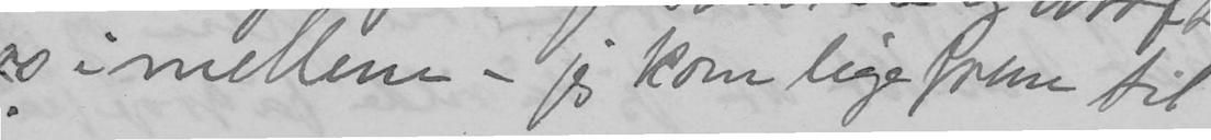os imellem - jeg kom ligefrem til
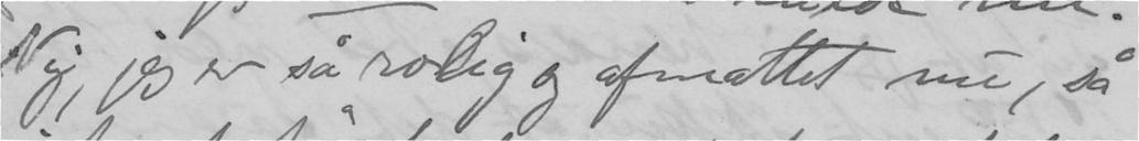Nej, jeg er så rolig og afmattet nu, så
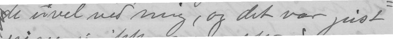de uvel ved mig, og det var just
